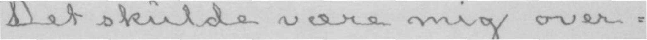Det skulde være mig over-
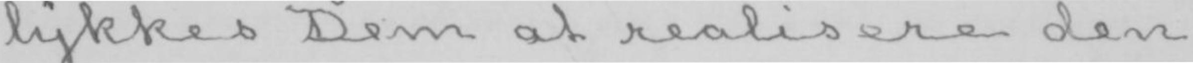lykkes Dem at realisere den
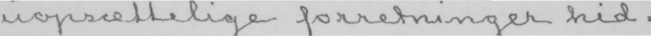uopsættelige forretninger hid-
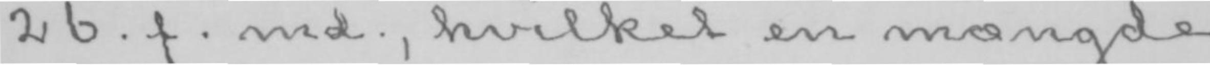26. f. md., hvilket en mængde
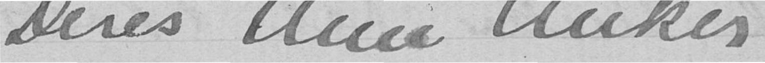Deres Nini Anker
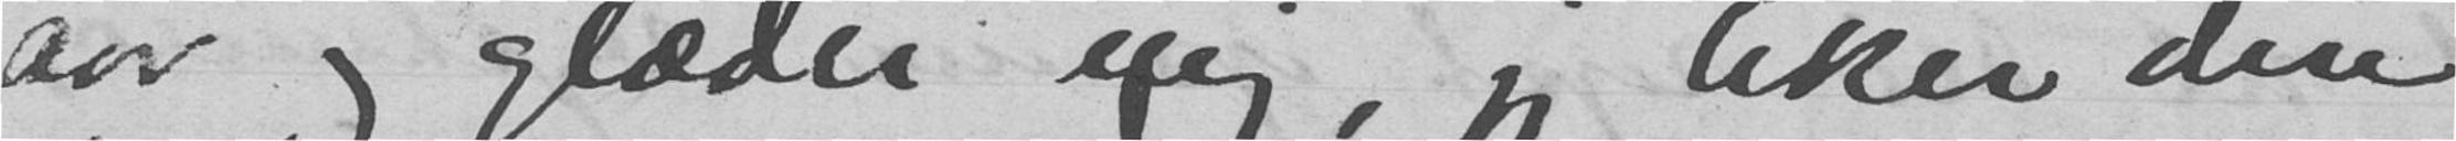aar og glæder mig, jeg liker den
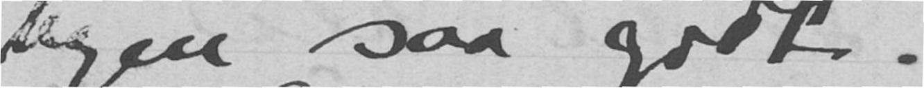byen saa godt.
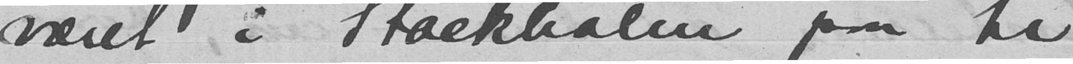været i Stockholm paa ti
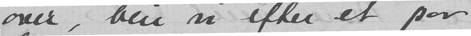over, blir vi efter et par
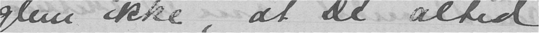glem ikke, at De altid
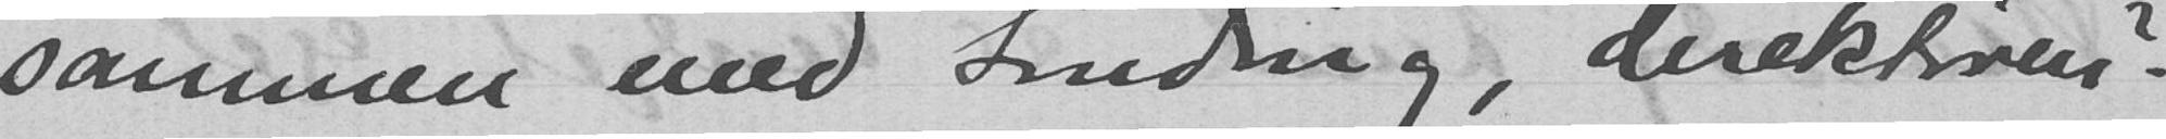sammen med Sinding, direktøren?
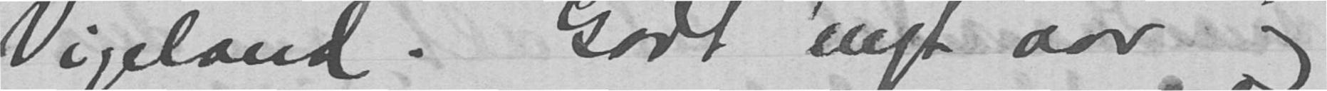Vigeland. Godt nyt aar og
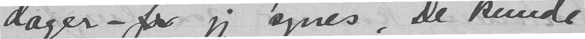dager - for jeg synes, De kunde
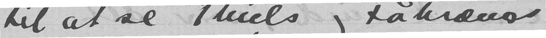til at se Thiels og Fåhræus
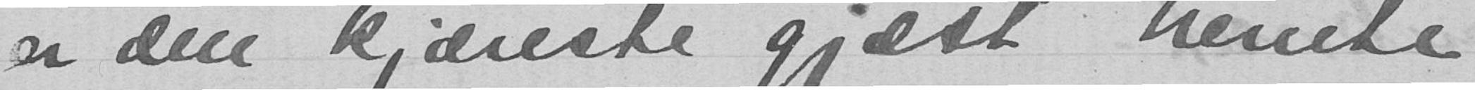er den kjæreste gjæst herute
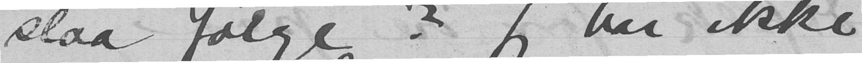slaa følge? Jeg har ikke
